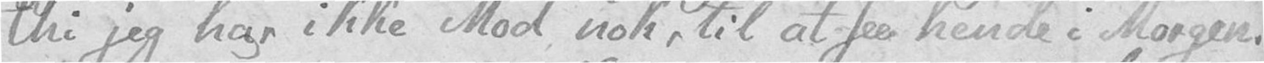thi jeg har ikke Mod nok, til at see hende i Morgen.
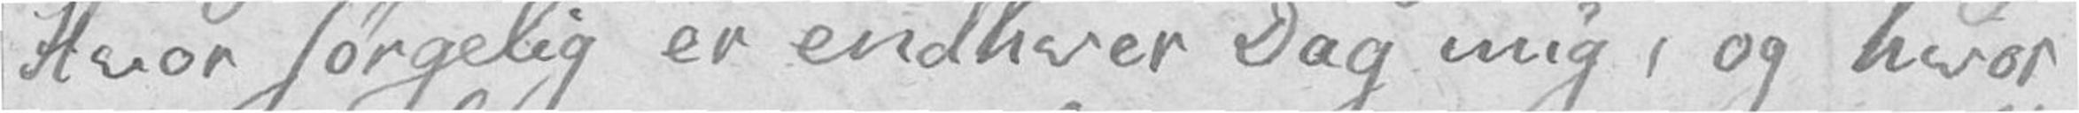Hvor sørgelig er endhver Dag mig, og hvor
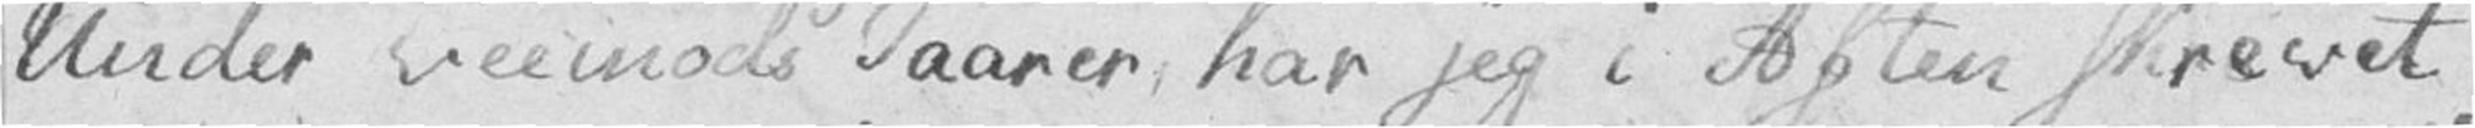Under veemods Taarer, har jeg i Aften skrevet
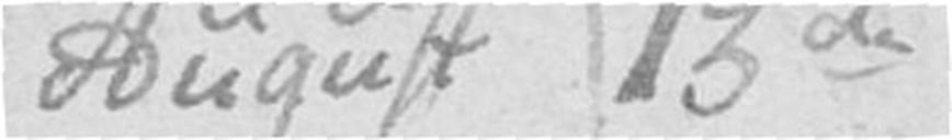August 13de
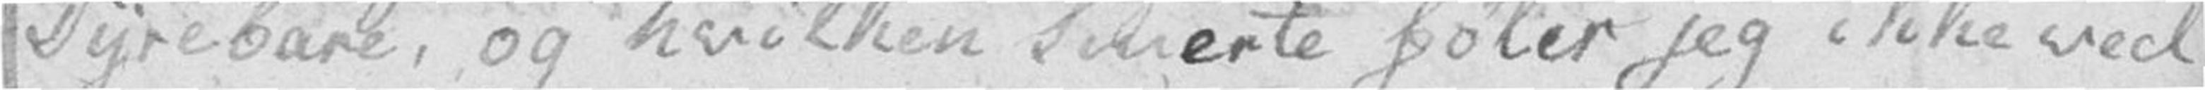Dyrebare, og hvilken Smerte føler jeg ikke ved
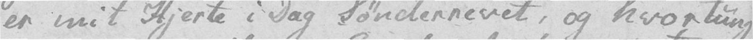er mit Hjerte i Dag Sønderrevet, og hvor tung
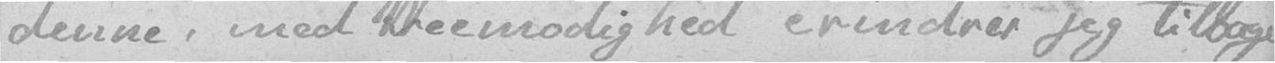denne, med Veemodighed erindrer jeg tilbage
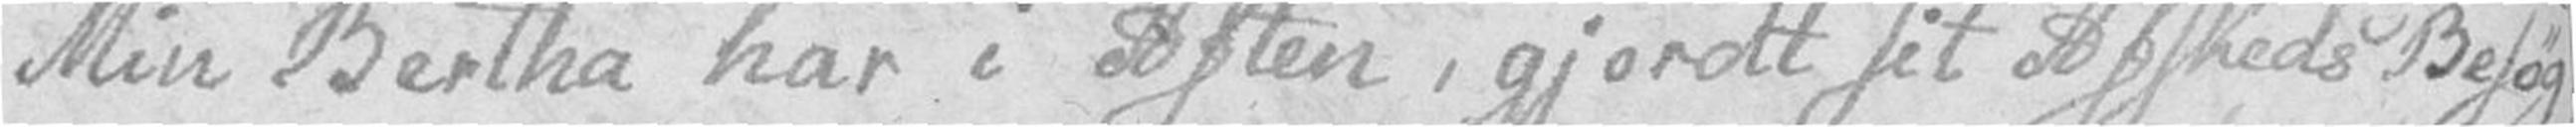Min Bertha har i Aften, gjordt til Afskeds Besøg
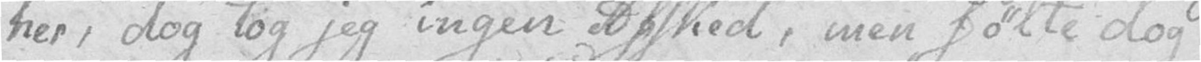her, dog tog jeg ingen Afsked, men følte dog
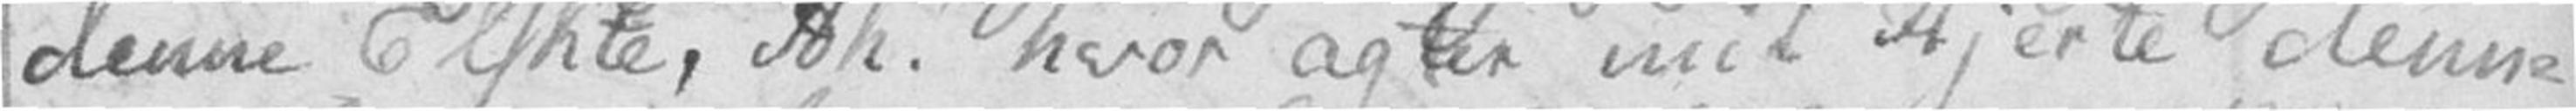denne Elskte, Ah! hvor agter mit Hjerte denne
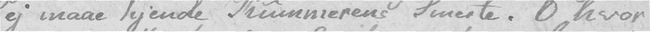ej maae kjende Kummerens Smerte. O hvor
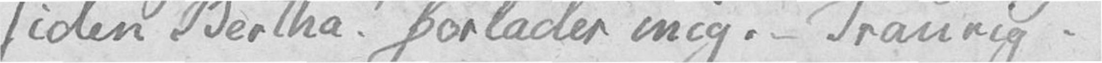siden Bertha! forlader mig. – Traurig.
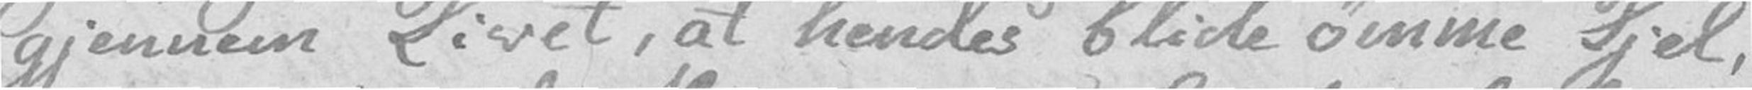gjennem Livet, at hendes blide ømme Sjel,
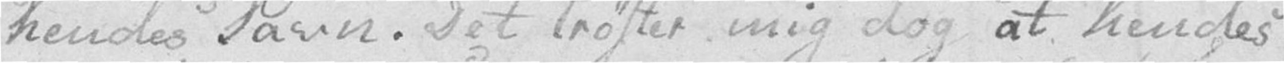hendes Savn. Det trøster mig dog at hendes
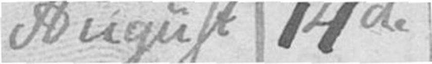August 14de
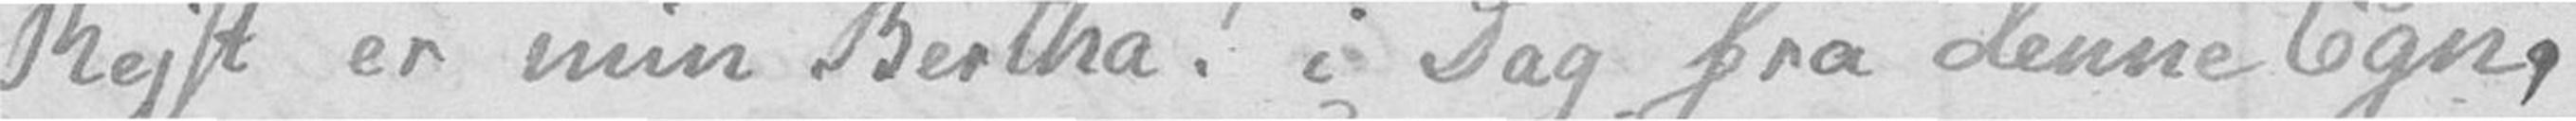Rejst er min Bertha! i Dag fra denne Egn,
